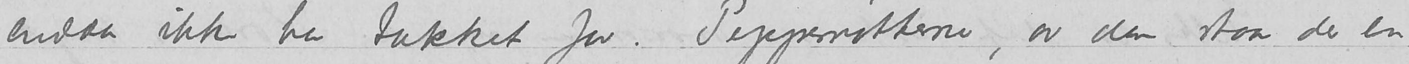endda ikke har takket for. Peppernøtterne, av dem staar der en
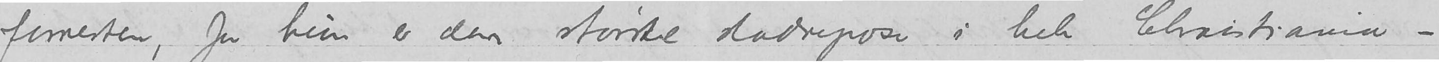forresten, for hun er den største sladrepose i hele Christiania –
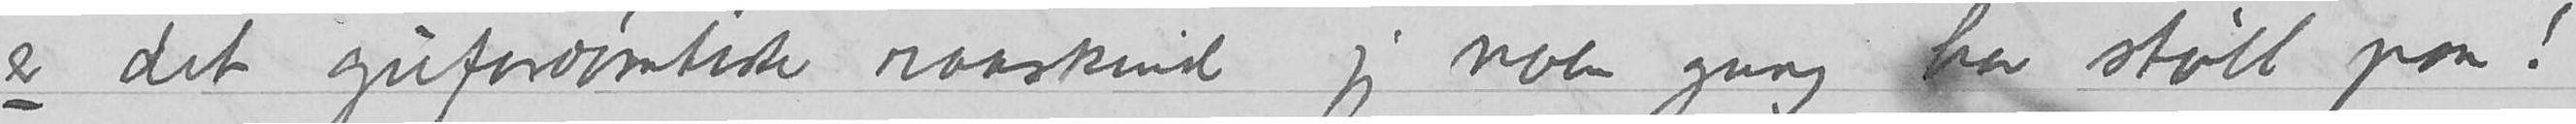er det gufordømteste raaskind jeg noen gang har støtt paa!
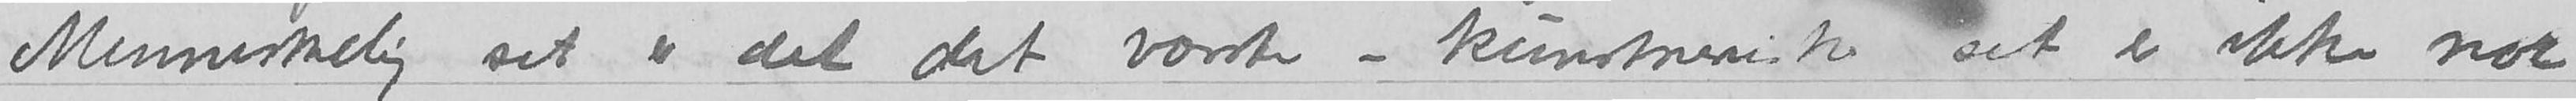Menneskelig set er det det værste – kunstnerisk set er ikke noe
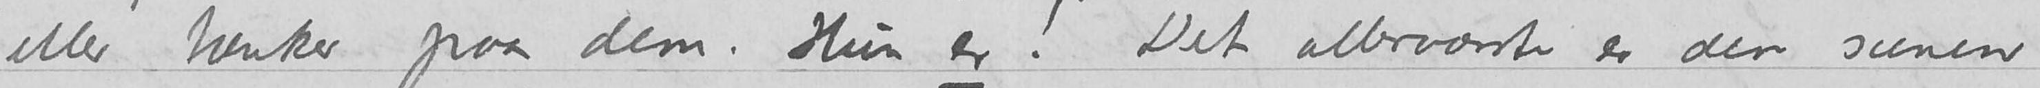eller tænker paa dem. Hun er! Det allerværste er den scenen
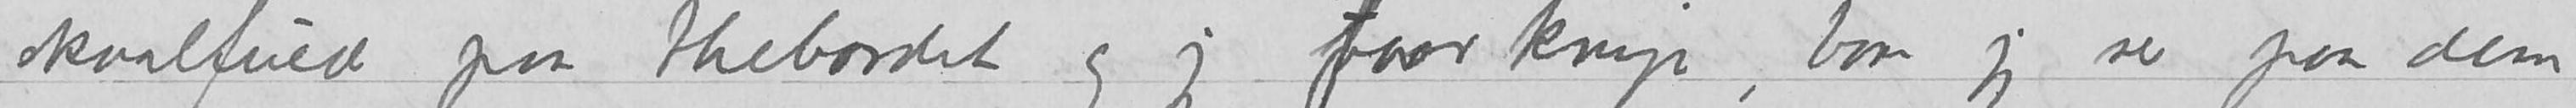skaalfuld paa thebordet og jeg faar knip, bare jeg ser paa dem
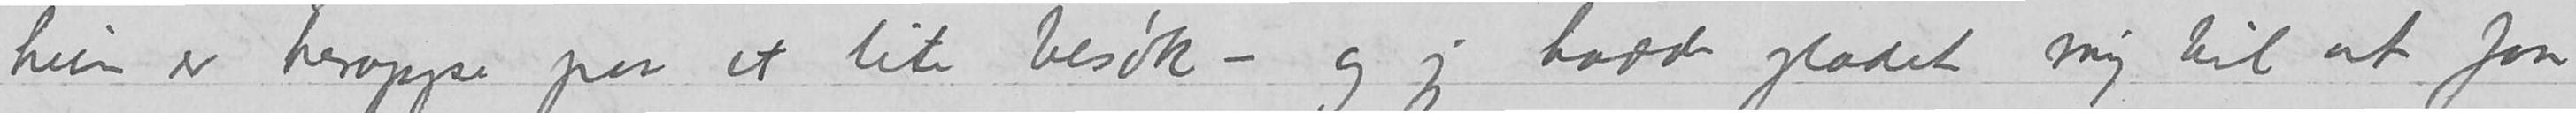hun er heroppe paa et lite besøk – og jeg hadde glædet mig til at faa
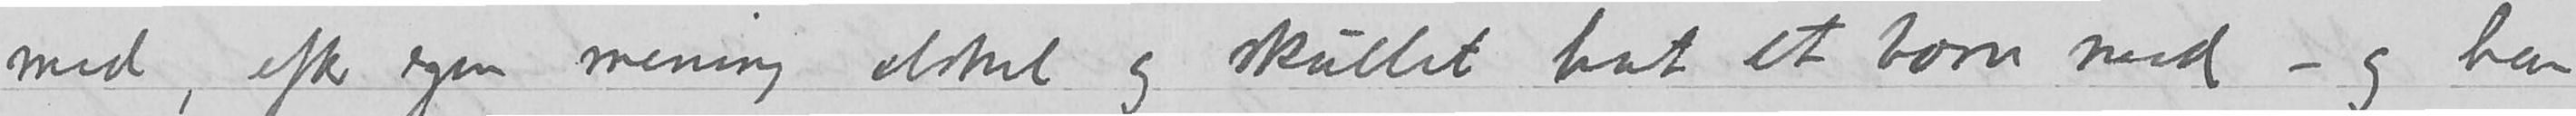med, efter egen mening elsket og skullet hat et barn med – og han
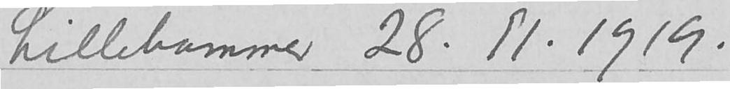Lillehammer 28.11.1919.
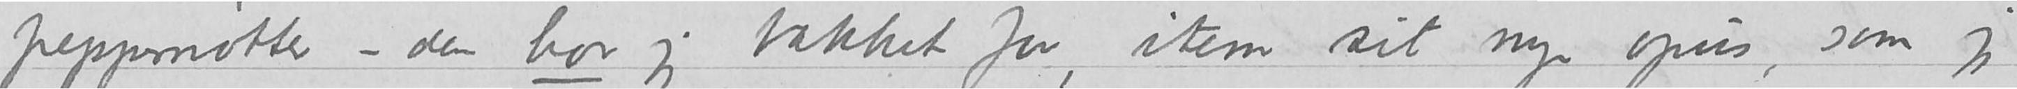peppernøtter – dem har jeg takket for, item sit nye opus, som jeg
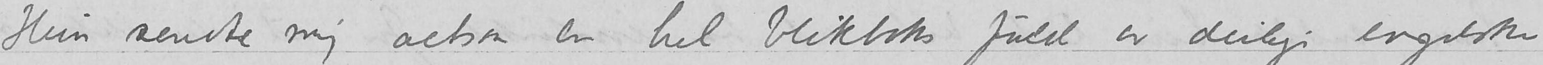Hun sendte mig altsaa en hel blikboks fuld av deilige engelske
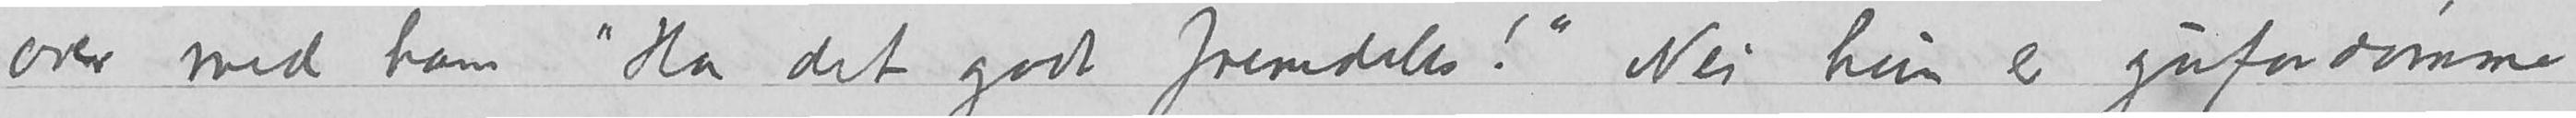arer med ham «Ha det godt fremdeles!» Nei hun er gufordømme
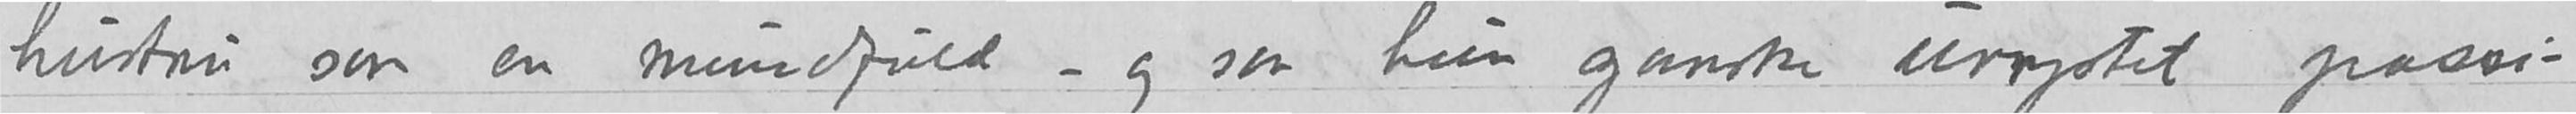hustru som en mundfuld – og som hun ganske urspotet passi
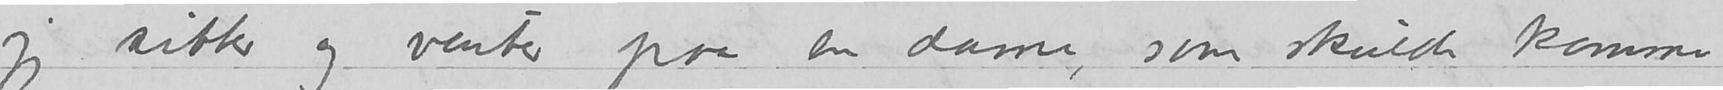jeg sitter og venter paa en dame, som skulde komme
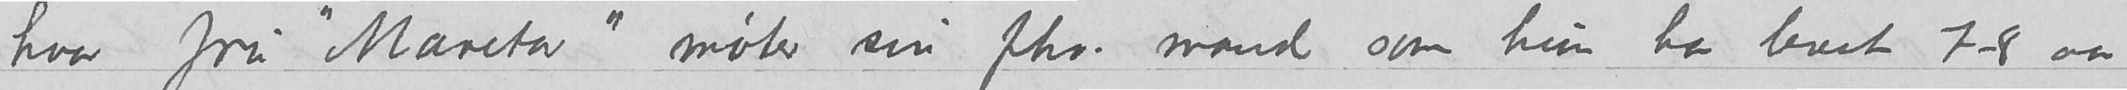hvor fru «Mareta» møter sin fhr. mand som hun har levet 7-8 aar
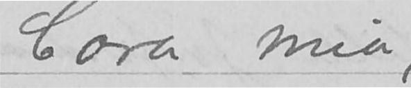Cora mia,
